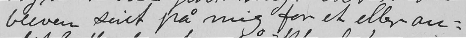bleven sint på mig for et eller an-
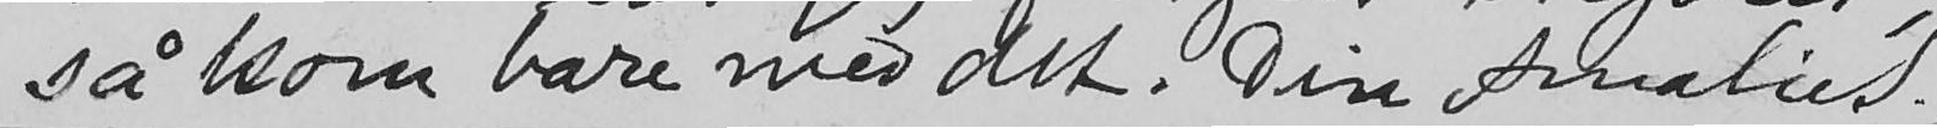så kom bare med det. Din Amalie S.
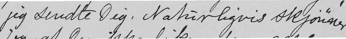jeg sendte Dig. Naturligvis skjønner
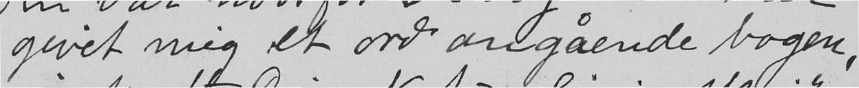givet mig et ord angående bogen,
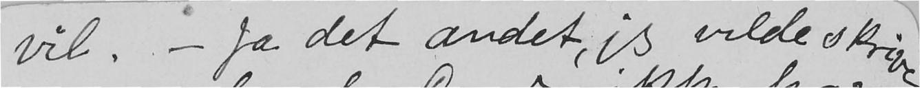vil. - Ja det andet, jeg vilde skrive
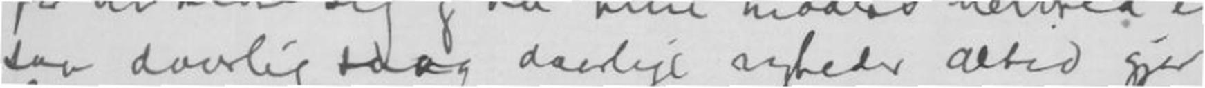saa daarlig idag daarlige nyheder altid gjør
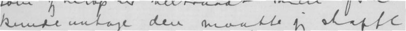kunde untage den maatte jeg skaffe
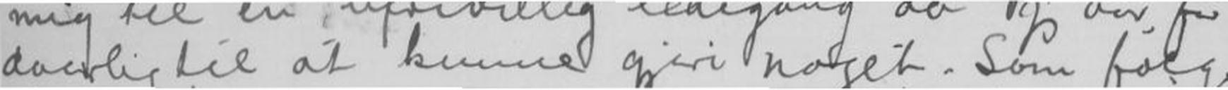daarlig til at kunne gjøre noget. Som følge
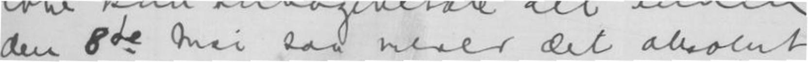den 8de Mai saa mener det absolut ruin
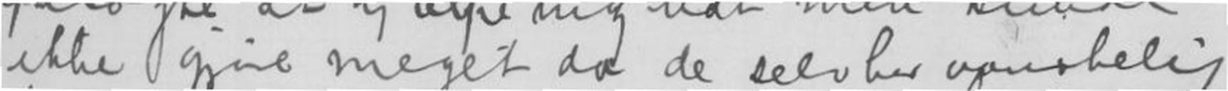ikke gjøre meget da de selv har vanskelig
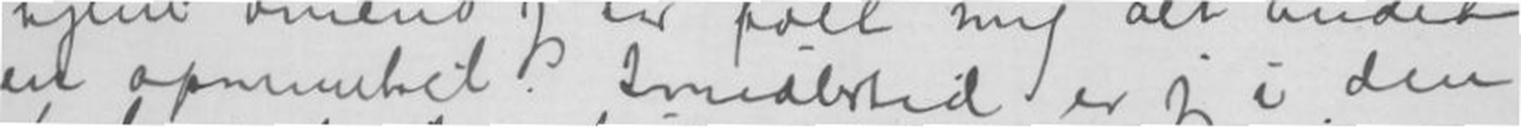en opmuntret. Imidlertid er jeg i den
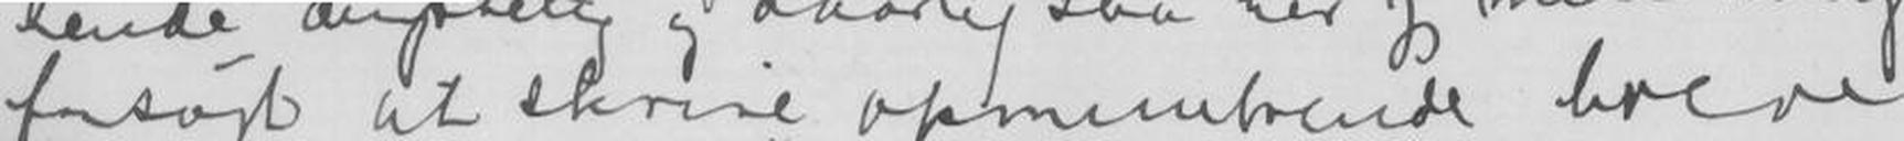forsøgt at skrive opmuntrende breve
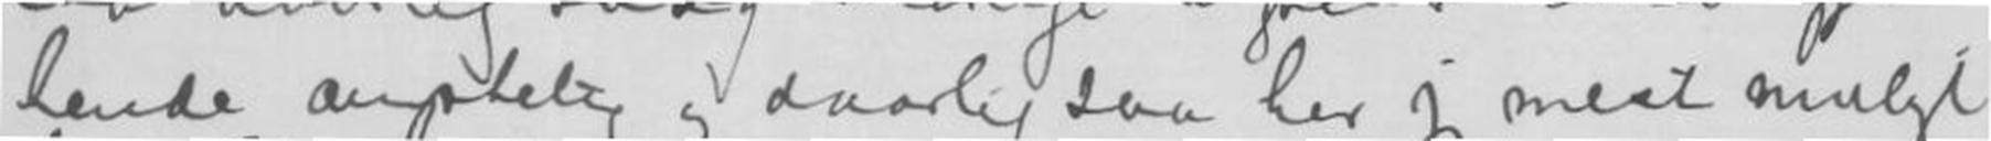hende ængstelig og daarlig saa har jeg mest muligt
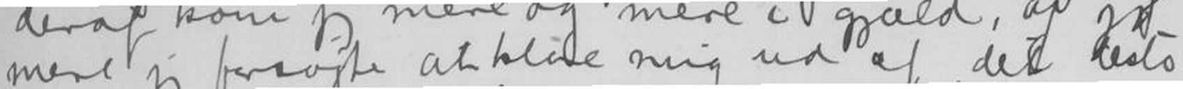mere jeg forsøgte at klare mig ud af det desto
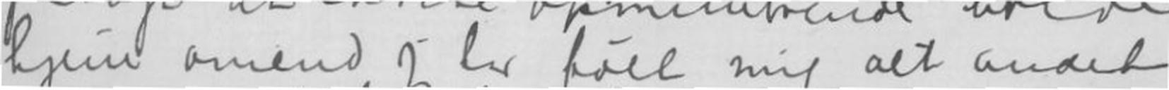hjem omend jeg har følt mig alt andet
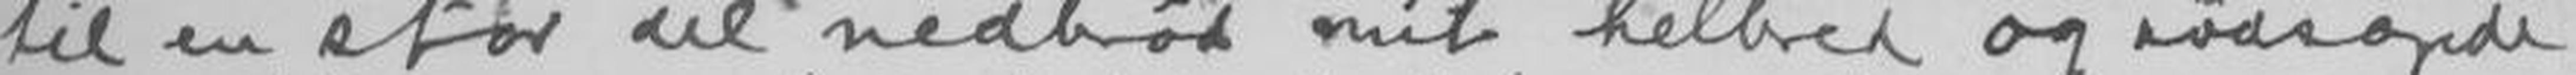til en stor del nedbrød mit helbred og nødsagde
